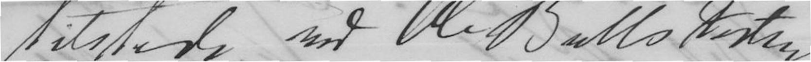tilstede ved Ole Bulls Festen
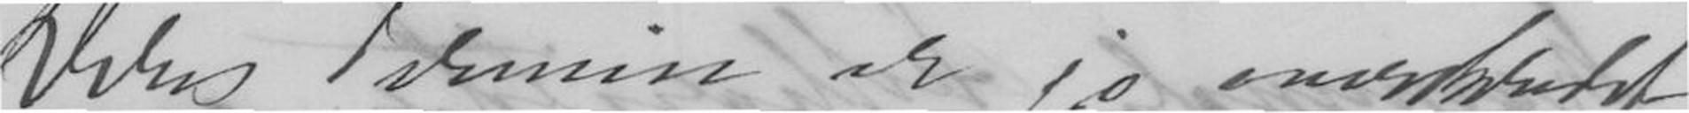Deres Termin er jo overskredet
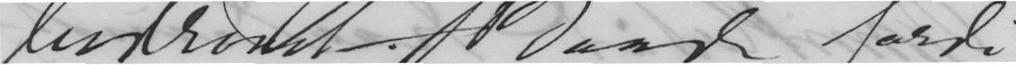bedrøvet. Baade fordi
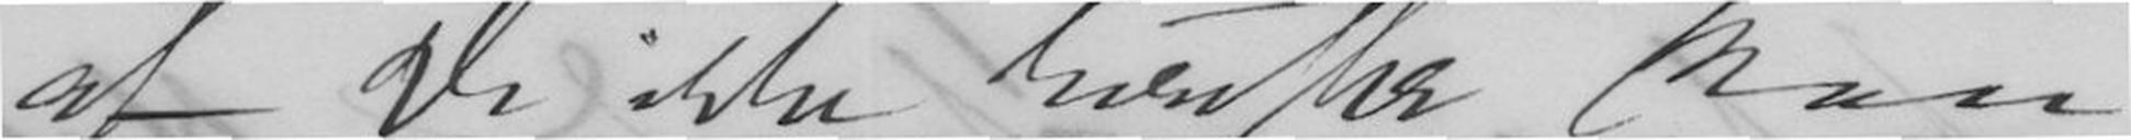at De ikke herefter kan
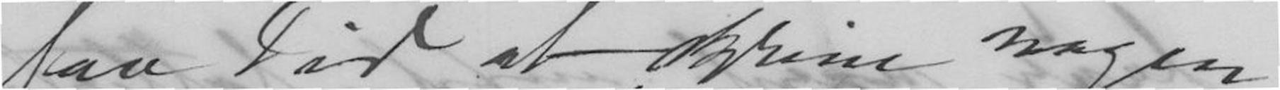faa Tid at skrive nogen
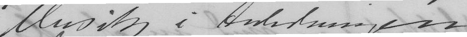Musik i Anledningen.
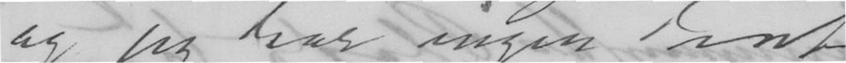og jeg har ingen Text
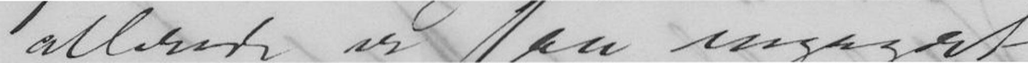allerede er saa engageret
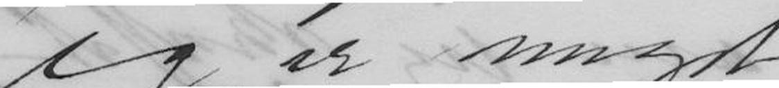Jeg er meget
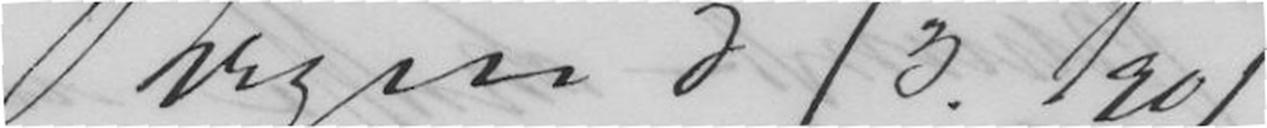Bergen 5/3. 1901
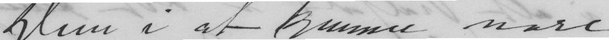Dem i at kunne være
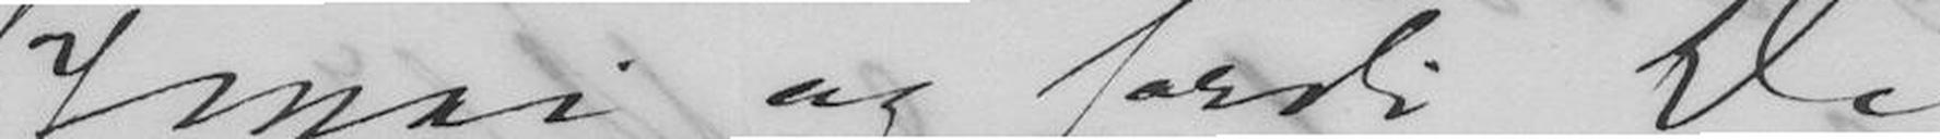17 Mai og fordi De
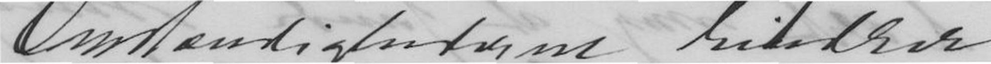Omstændighederne hindrer
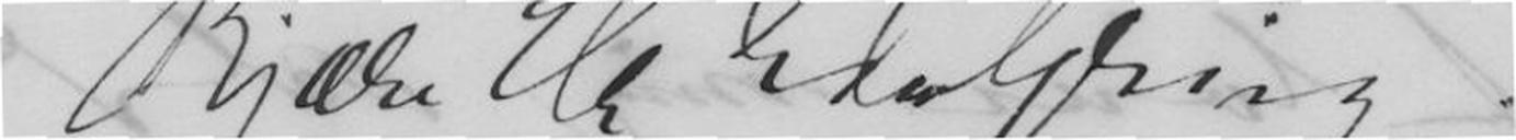Kjære Hr Edv Grieg!
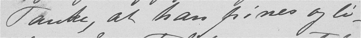Tanke, at han fines og li-
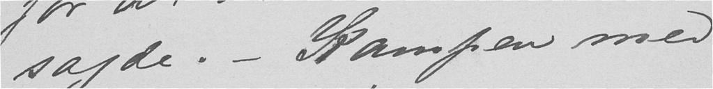sagde. - Kampen med
